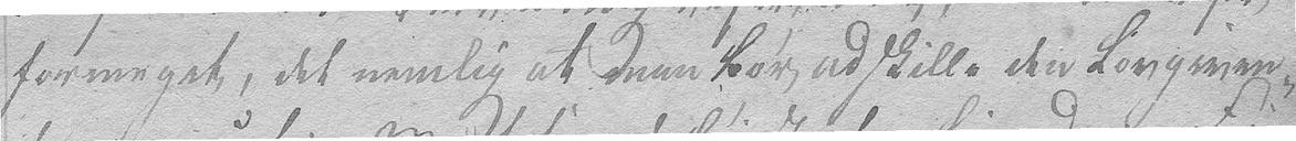formeget, det nemlig at denne bør udskille den lovgiven-
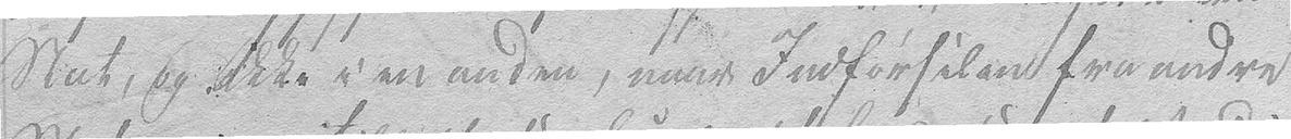Stat, og ikke i en anden, naar Indførselen fra andre
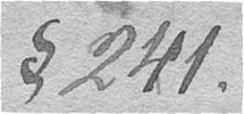§ 241.
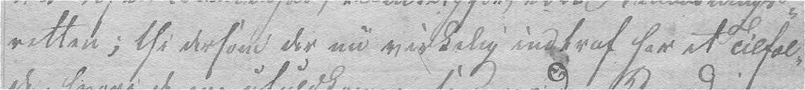retten; thi dersom der nu virkelig indtraf her et Tilfæl-
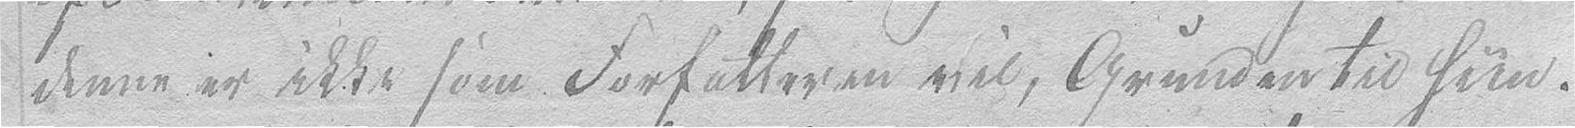denne er ikke som Forfatteren vil, Grunden til hiin.
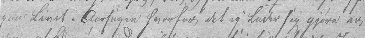paa Livet. Aarsagen hvorfor det ey lader sig gjøre er
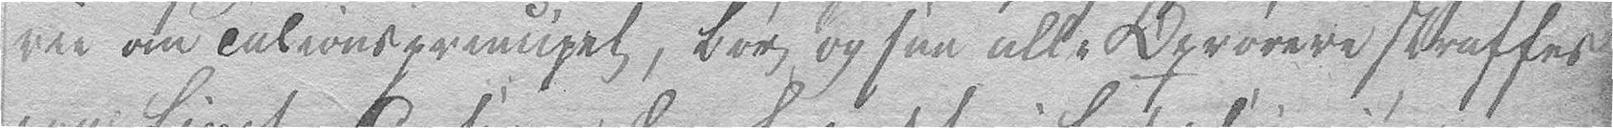rie om Talionsprincipet, bør ogsaa alle Oprørere straffes
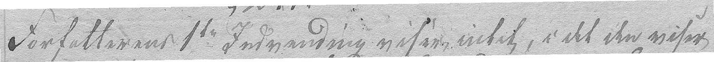Forfatterens 1te Indvending viser intet, i det den viser
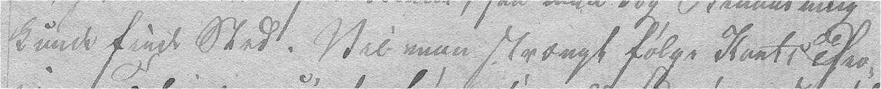kunde finde Sted. Vil man strængt følge Kants Theo-
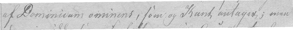af Dominium eminens, som og Kant antager, men
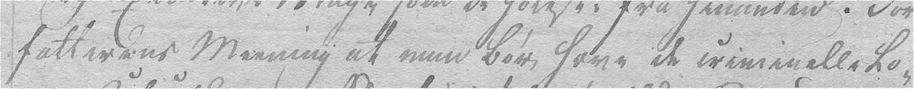fatterens Meening at man bør hæve de criminelle Lo-
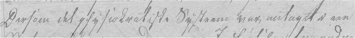Dersom det physiokratiske System var antaget i en
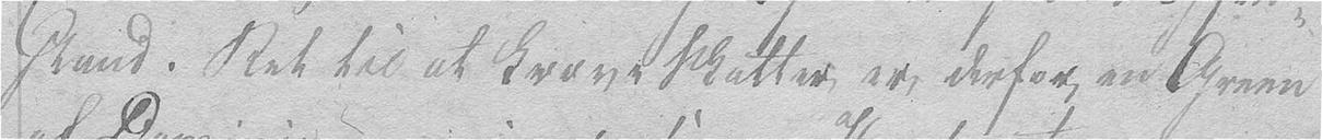stand. Ret til at kræve Skatter er derfor en Green
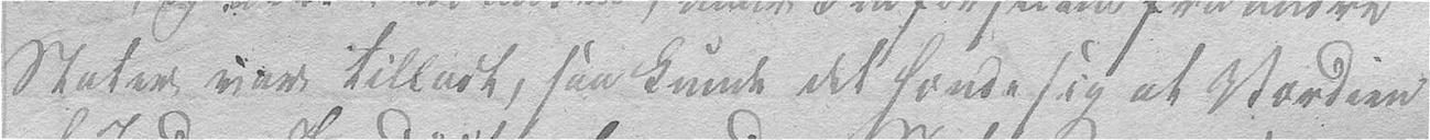Stater var tilladt, saa kunde det hænde sig at Værdien
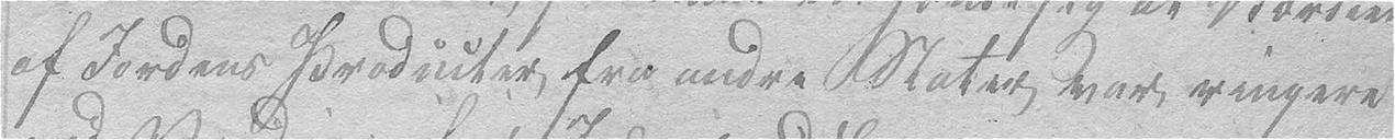af Jordens Producter fra andre Stater var ringere
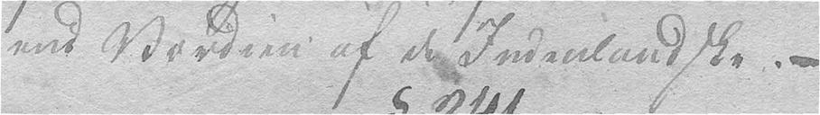end Værdien af de Indenlandske. –
